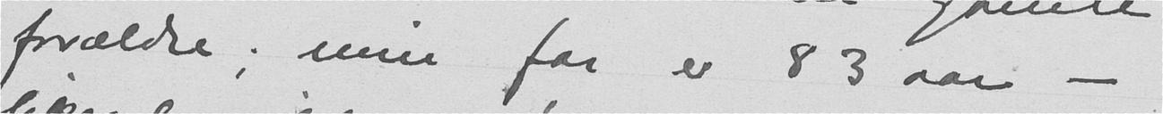forældre; min far er 83 aar -
